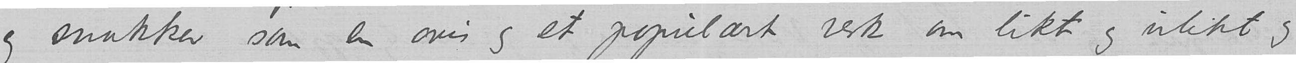og snakker som en avis og et papirlært verk om likt og ulikt og
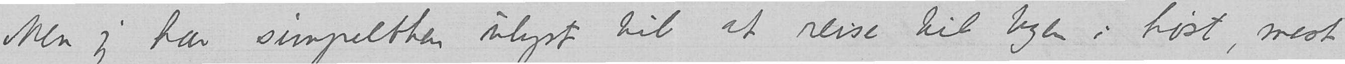Men jeg har simpelthen ulyst til at reise til byen i høst, mest
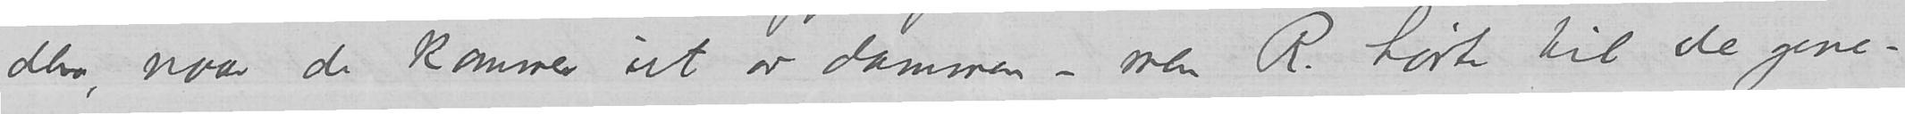dem, naar de kommer ut av dammen – men R. hørte til de gene-
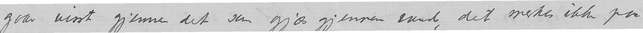gaar visst gjennem det som gjæs gjennem vand, det merkes ikke paa
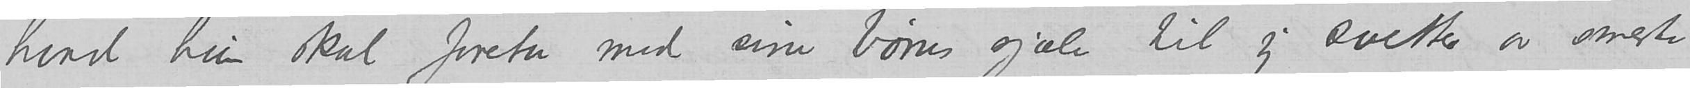hvad hun skal foreta med sine børns sjæle til jeg svetter av smerte
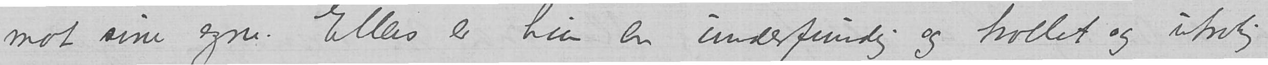mot sine egne. Ellers er hun en underfundig og trollet og utrolig
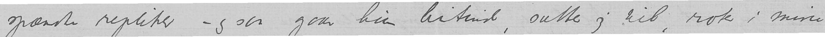spændte repliker – og saa gaar hun hitind, sætter sig til, roter i mine
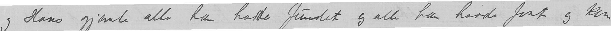og Hans gjemte alle han hadde fundet og alle han hadde faat og kom
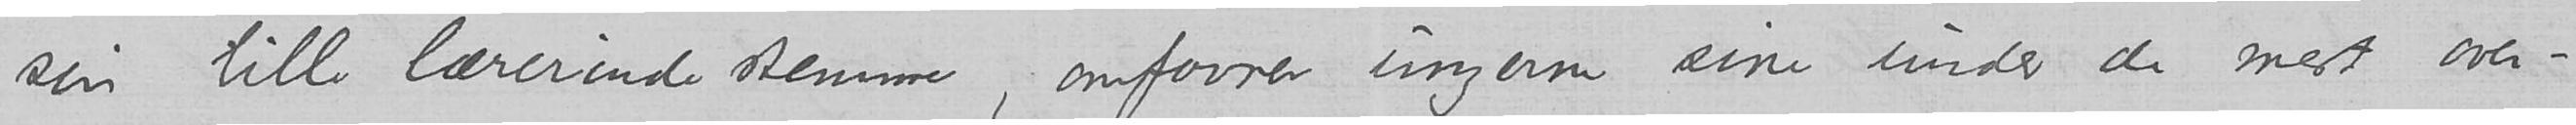sin lille lærerindestemme, omfavner ungerne sine under de mest over-
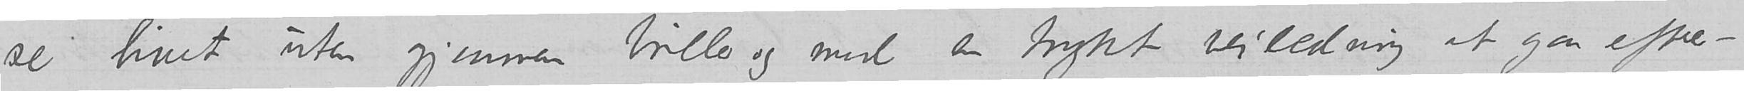se livet uten gjennem briller og med en trykt veiledning at gaa efter –.
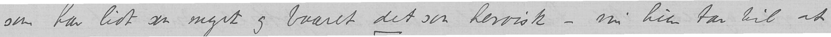som har lidt saa meget og baaret det saa heroisk – nu hun tar til at
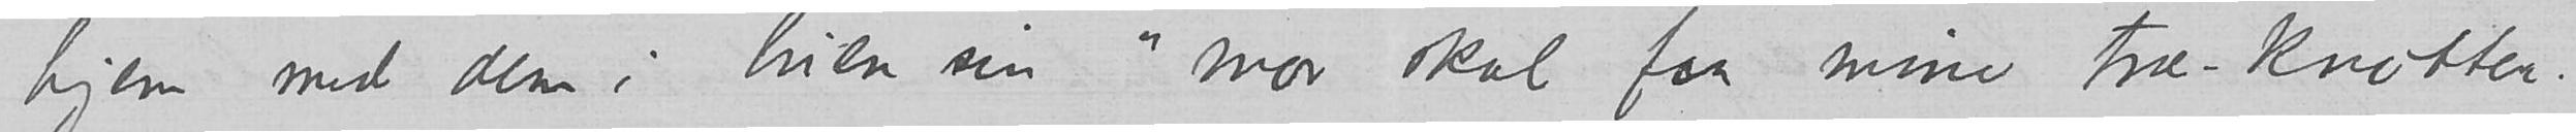hjem med dem i luen sin «mor skal faa mine træ-knotter».
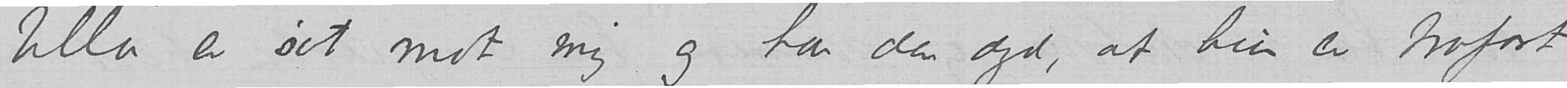Ulla er søt mot mig og har den dyd, at hun er trofast
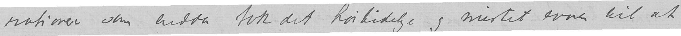rationer som endda tok det høitidelige og mistet evnen til at
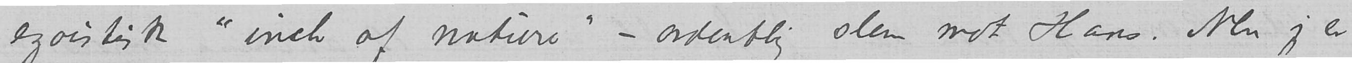egoistisk «inch of nature» – ordentlig slem mot Hans. Men jeg er
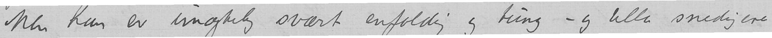Men han er unægtelig svært enfoldig og tung – og Ulla snedigere
